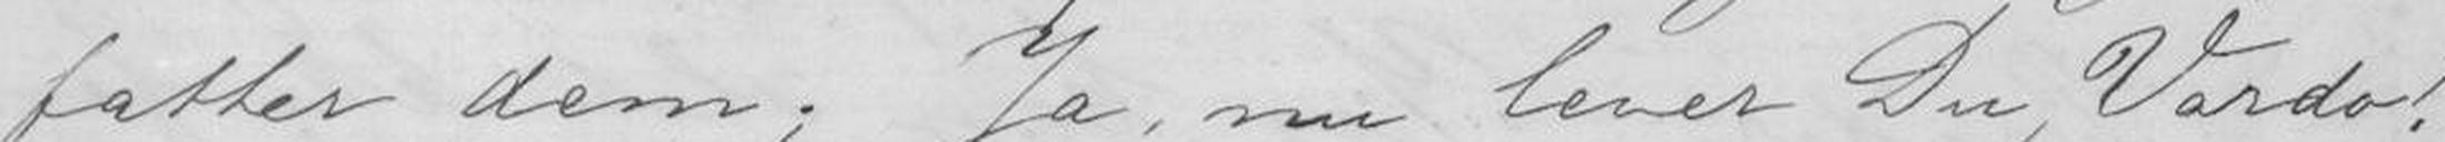fatter dem; Ja, nu lever Du, Vardo!
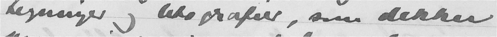tegninger og litografier, som dekker
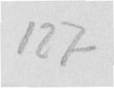127
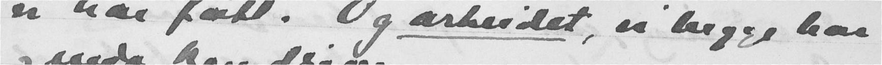vi har fått. Og arbeidet, vi begge har
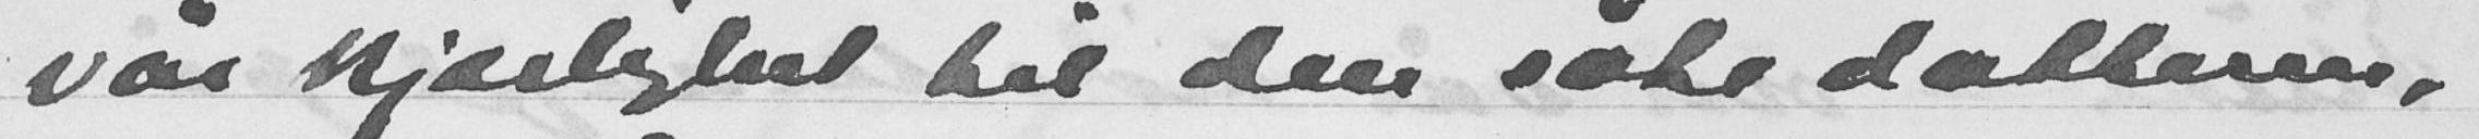vår kjærlighet til den søte datteren,
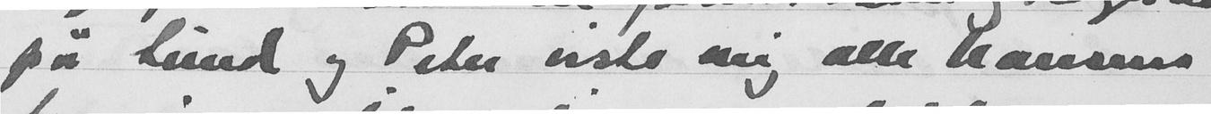på Lund og Peter viste om alle Nansens
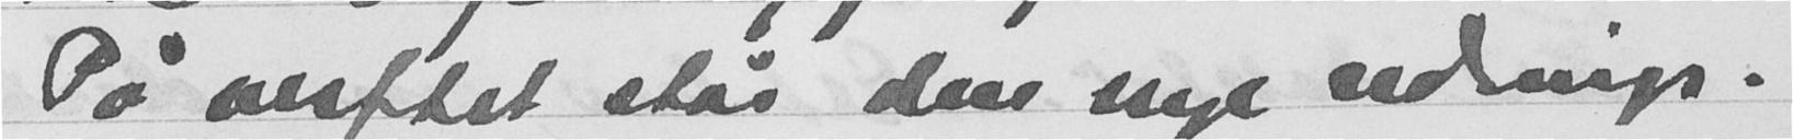På verftet står den nye rednings-
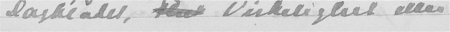Dagbladet,  Virkelighet eller
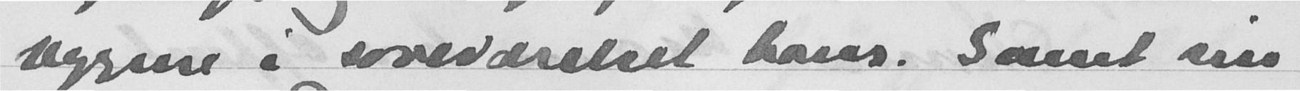veggene i soveværelset hans. Samt sin
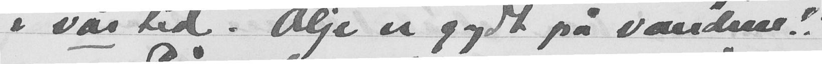i vår tid. Olje er gydt på vandene!!
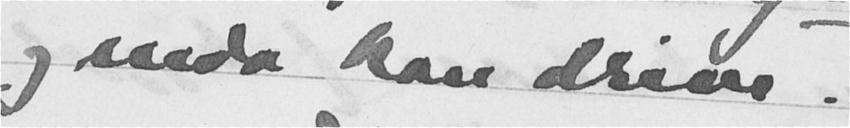og enda kan drive.
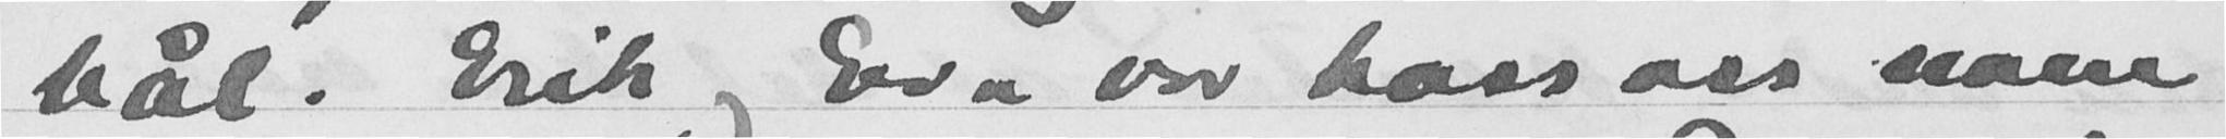bål. Erik og Eva var hoss oss noen
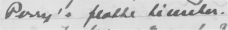Perry's flotte timeter.
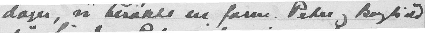dager, vi besøkte en favn. Peter og Borghild
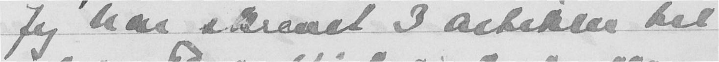Jeg har skrevet 3 Artikler til
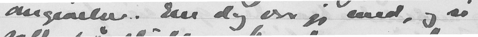omgivelser. En dag var jeg med, og vi
